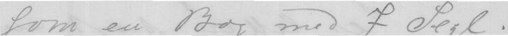som en Bog med 7 Segl.
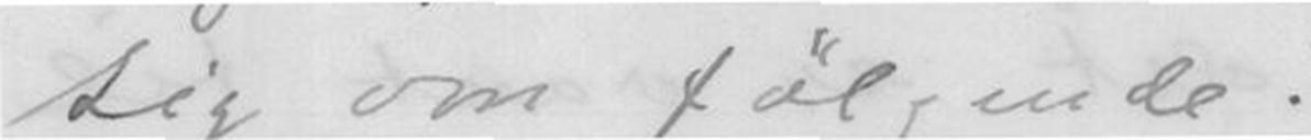sig om følgende:
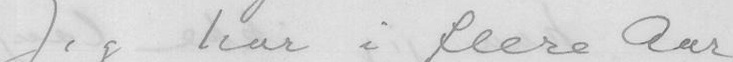Jeg har i flere Aar
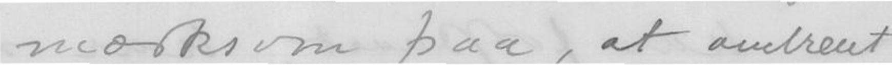merksom paa, at omtrent
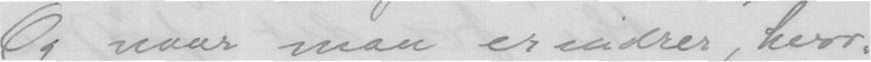Og naar man erindrer, hvor-
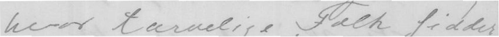hvor tarvelige Folk sidder
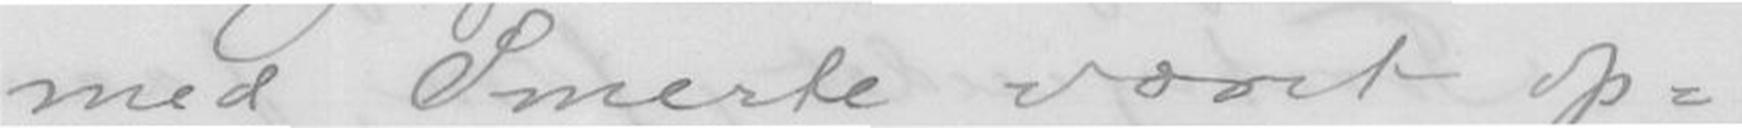med Smerte været op-
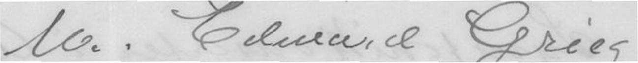Hr. Edward Grieg!
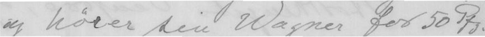og hører sine Wagner for 50 Ptz.
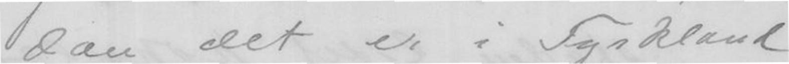dan det er i Tyskland
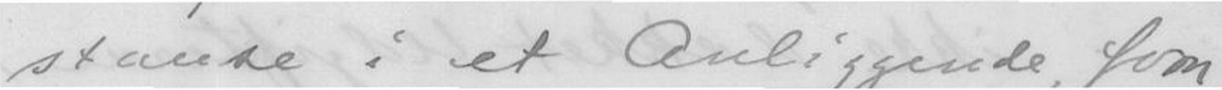stanse i et Anliggende, som
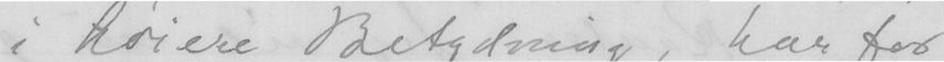i Høiere Betydning, har for
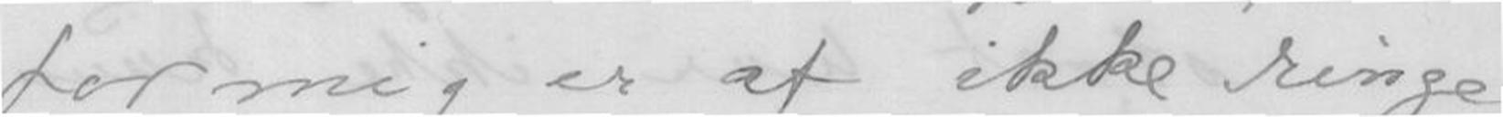for mig er af ikke ringe
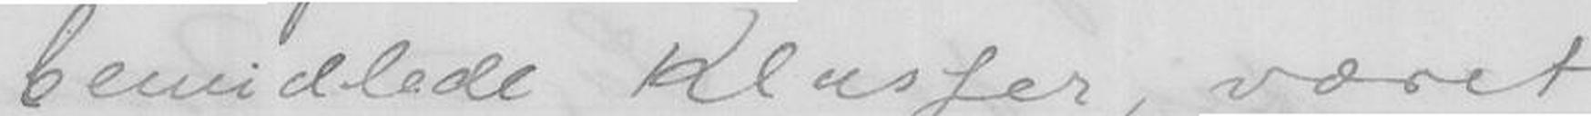bemidlede Klasser, været
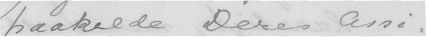paakalde Deres Assi-
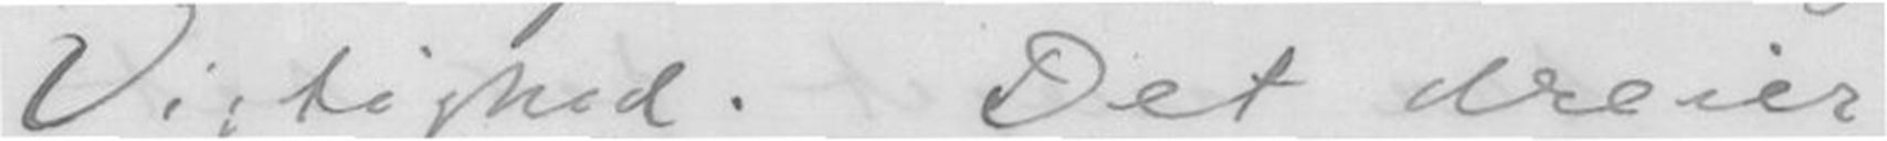Vigtighed. Det dreier
